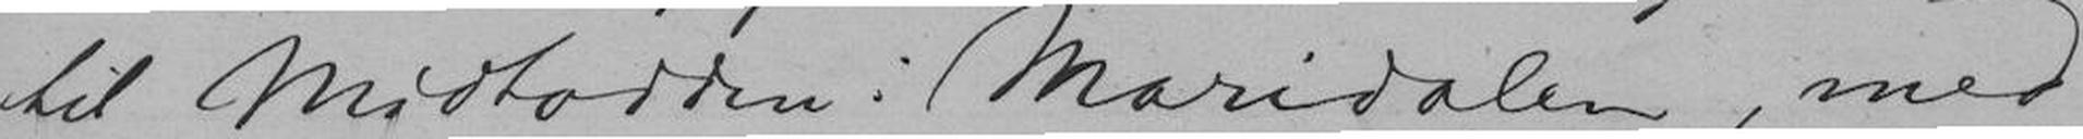til Midtodden i Maridalen, med
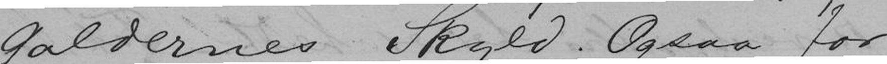Galdernes Skyld. Ogsaa for
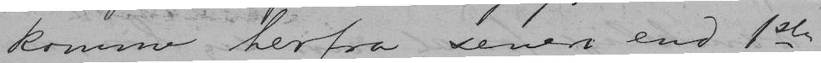komme herfra senere end 1ste
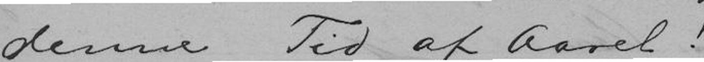denne Tid af Aaret!
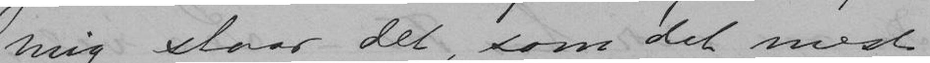mig staar det, som det mest
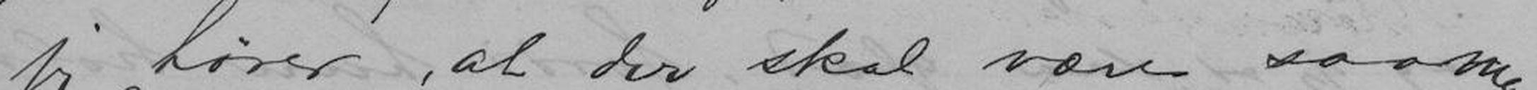jeg hører, at der skal være saameget
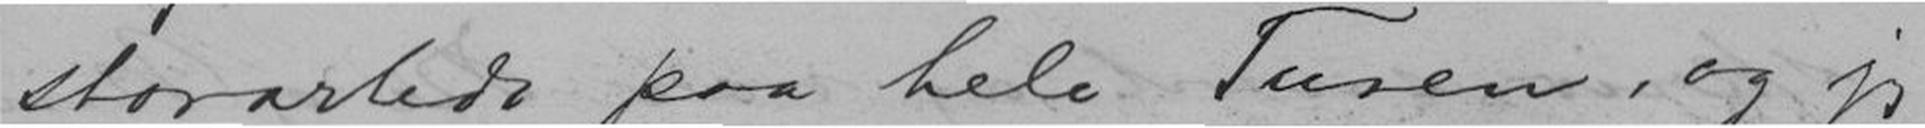storartede paa hele Turen, og jeg
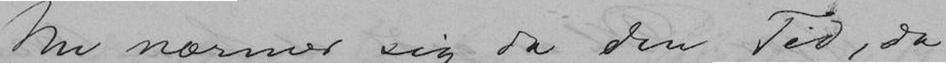Nu nærmer sig da den Tid, da
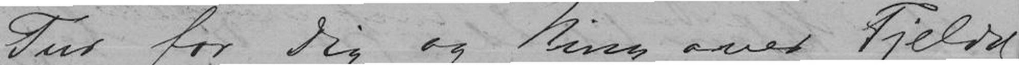Tur for Dig og Nina over Fjeldet,
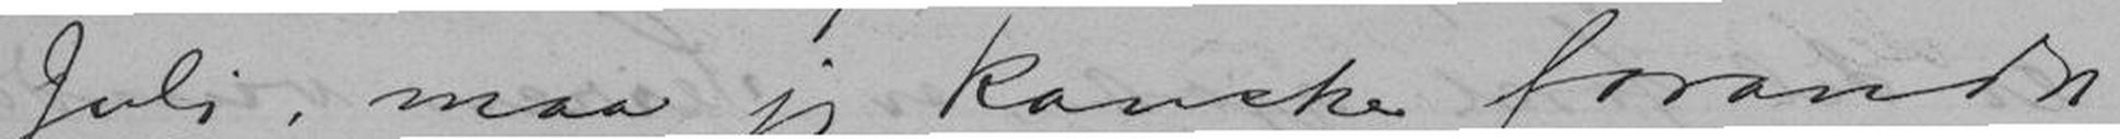Juli, maa jeg kanske forandre
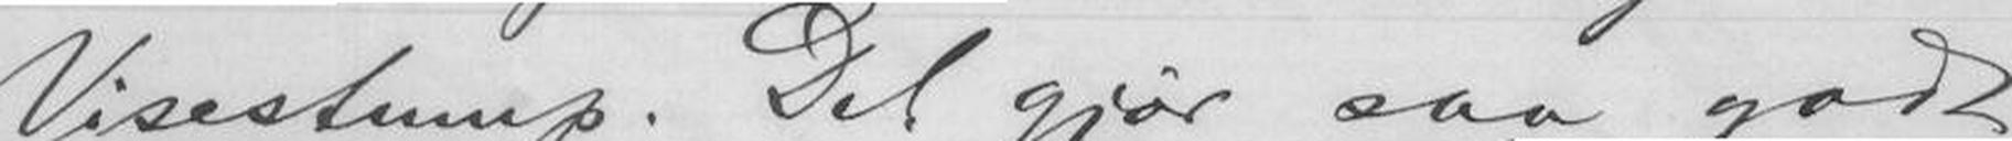Visestump. Det gjør saa godt
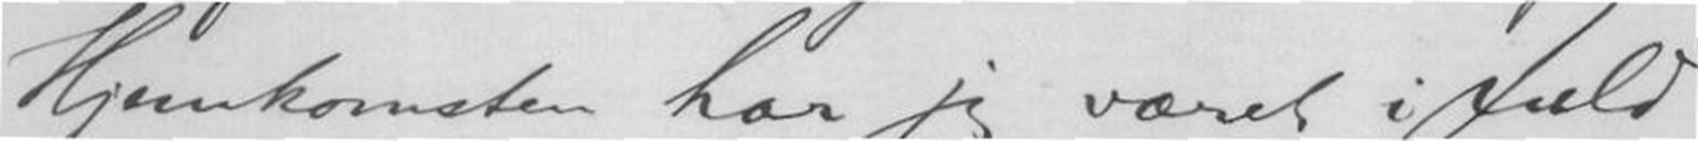Hjemkomsten har jeg været i fuld
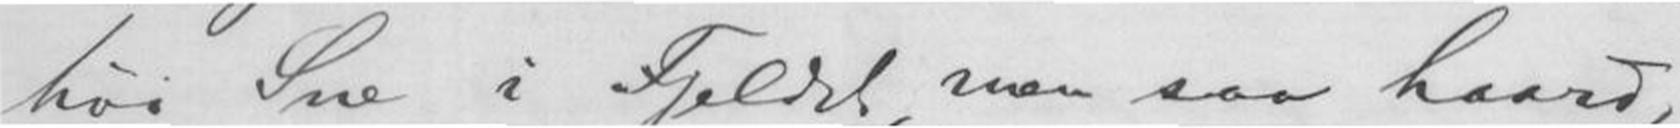høi Sne i Fjeldet, men saa haard,
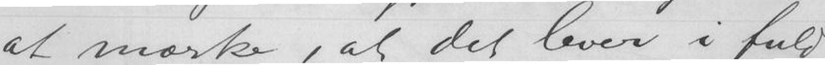at mærke, at det lever i fuld
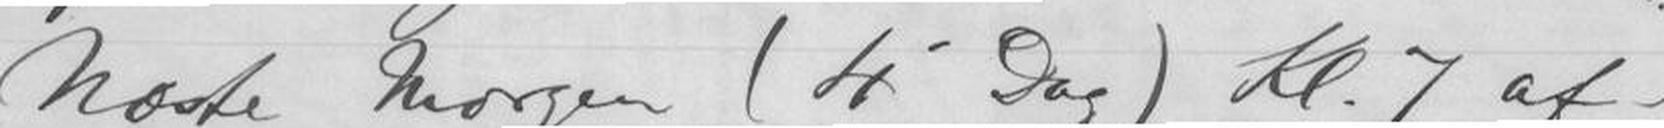Næste Morgen (4de Dag) kl.7 af-
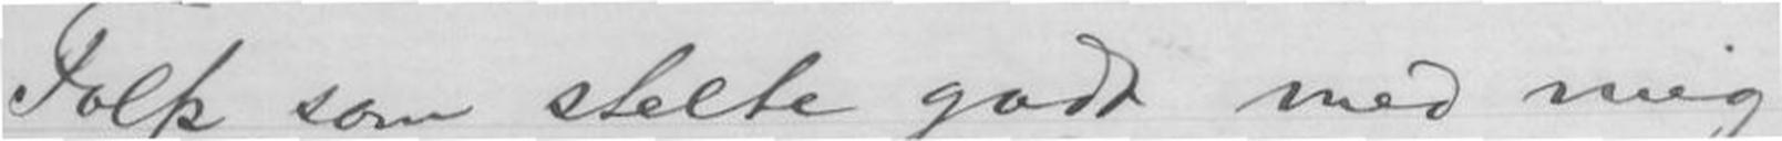Folk, som stelte godt med mig
